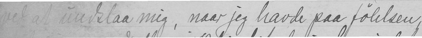ved at undslaa mig, naar jeg havde paa følelsen,
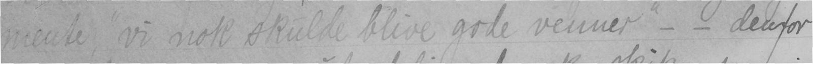mente "vi nok skulde blive gode venner" - - ? derfor
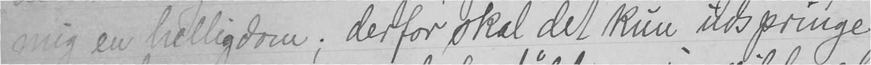mig en helligdom; derfor skal det kun udspringe
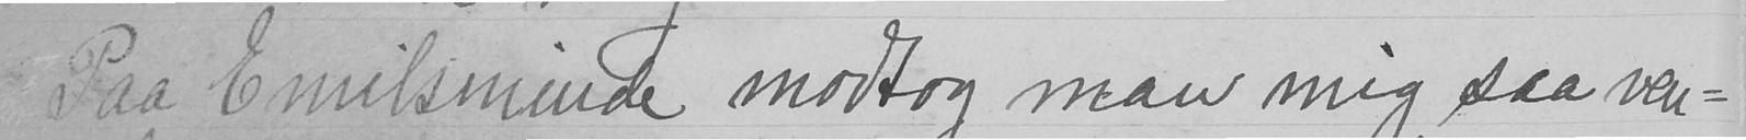Paa Emilsminde modtog man mig saa ven-
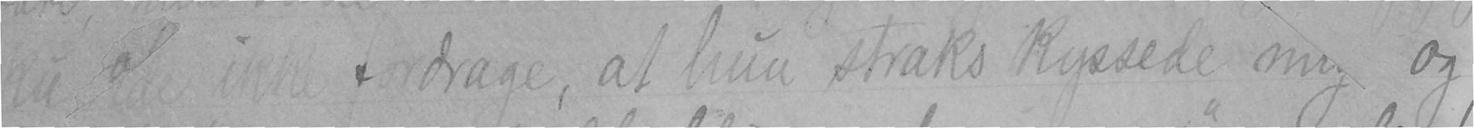nu  ikke fordrage, at hun straks kyssede mig og
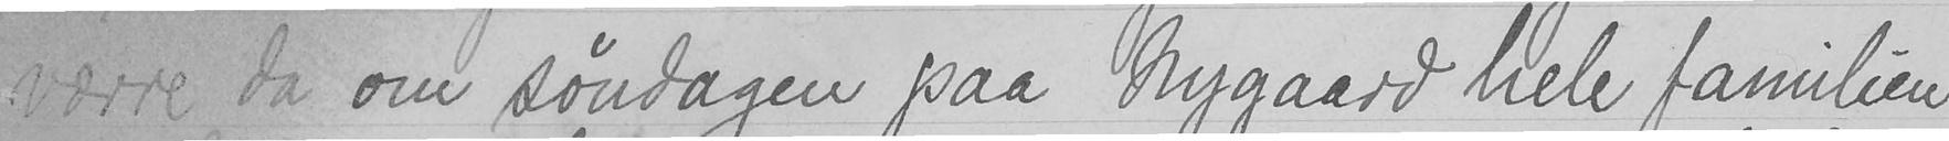værre da om søndagen paa Nygaard hele familien
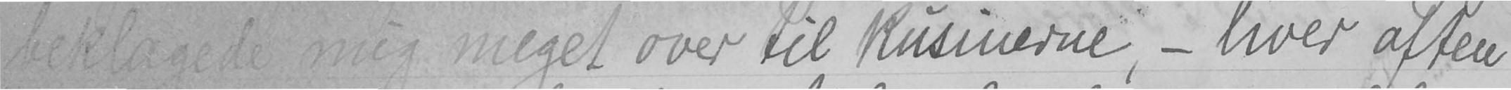beklagede mig meget over til kusinerne, - hver aften
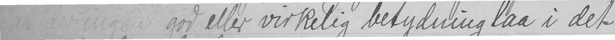at der ingen god eller virkelig betydning laa i det
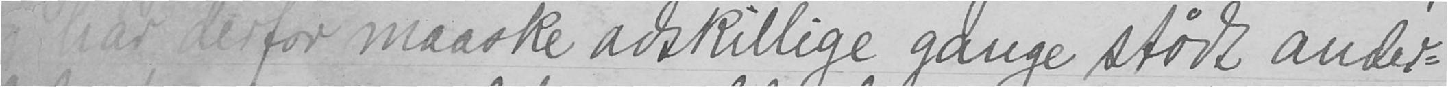har derfor maaske adskillige gange stødt ander-
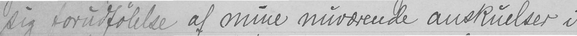sig forudfølelse af mine nuuværende anskuelser i
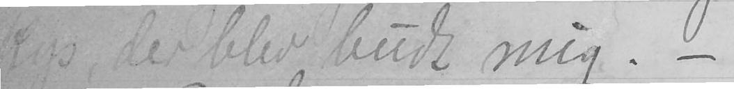kys, der blev budt mig. -
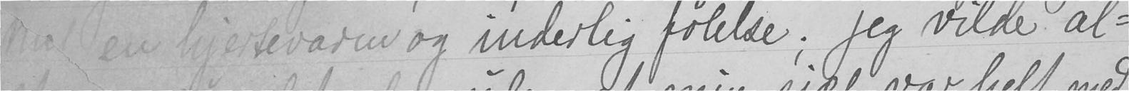med en hjertevarm og underlig følelse; jeg vilde al-
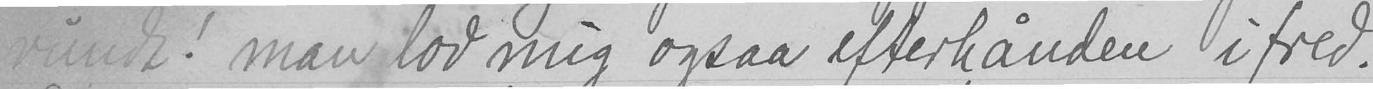rundt! man lod mig ogsaa efterhanden ifred.
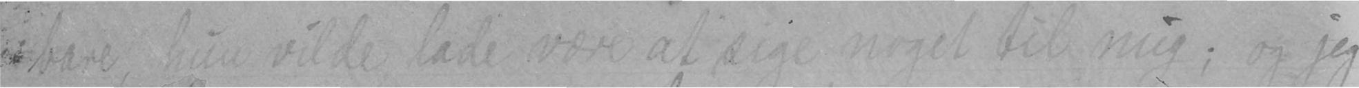bare, hun vilde lade være at sige noget til mig; og jeg
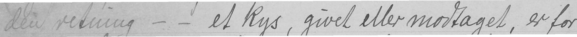den retning - - et kys, givet eller modtaget, er for
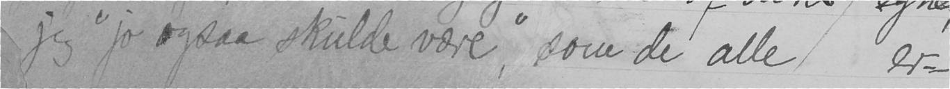jeg "jo ogsaa skulde være," som de alle er-
